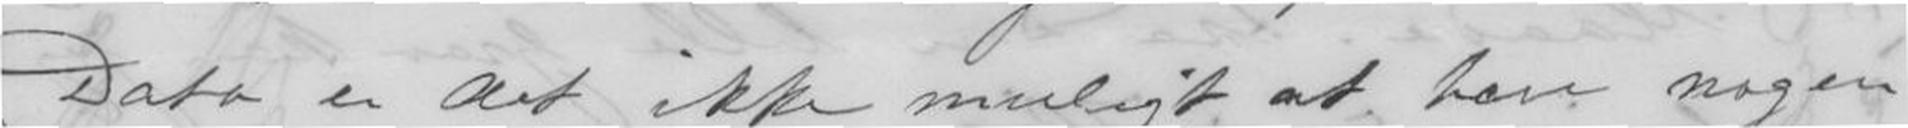Dato er det ikke muligt at have nogen
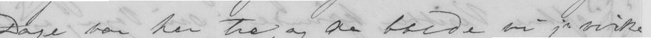Dage var her tre, og da boede vi virke-
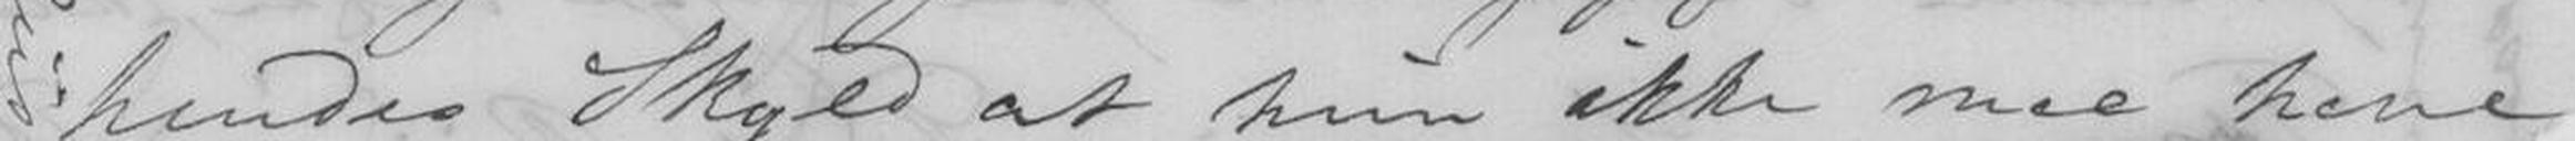hendes Skyld at hun ikke maa have
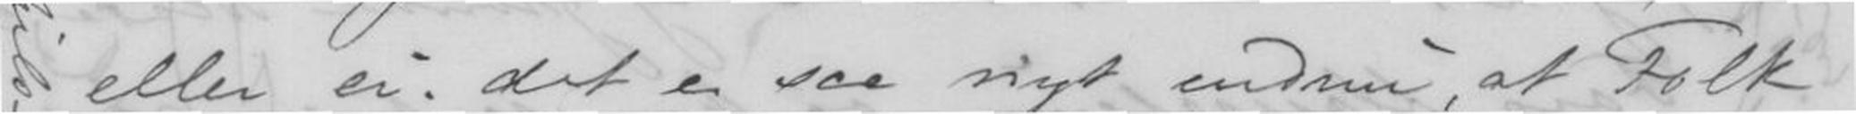eller ei; det er saa nyt endnu, at Folk
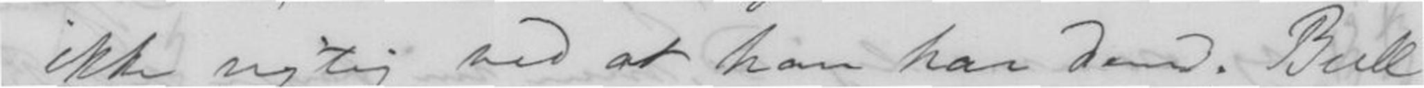ikke rigtig ved at han har dem; Beek
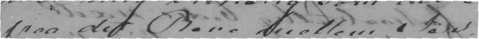paa det Rene mellem Jer. -
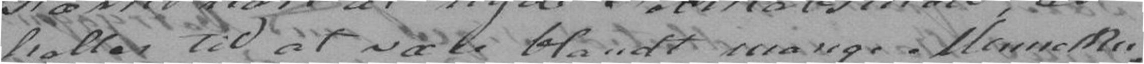heller til at være blandt mange Mennesker,
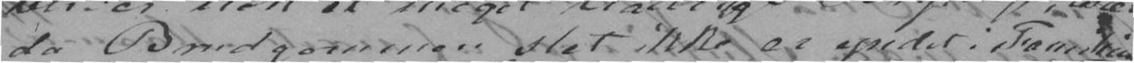da Brudgommen slet ikke er yndet i Familien.
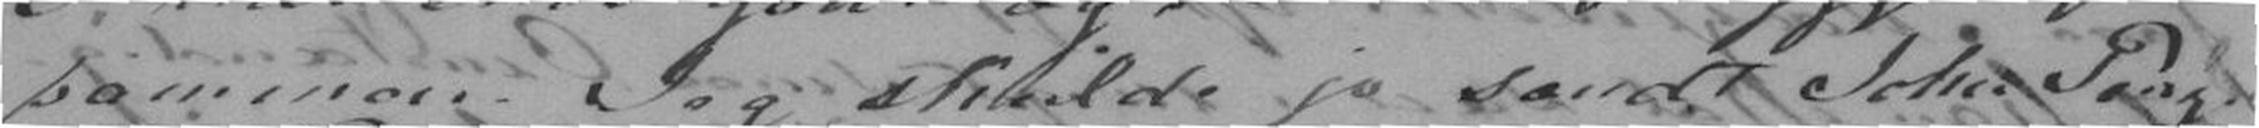sammen. Jeg skulde jo sendt John Penge
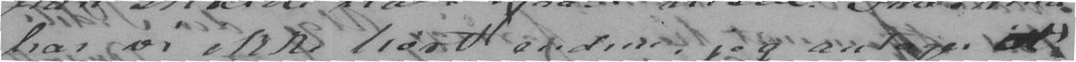har vi ikke hørt endnu, jeg antager at
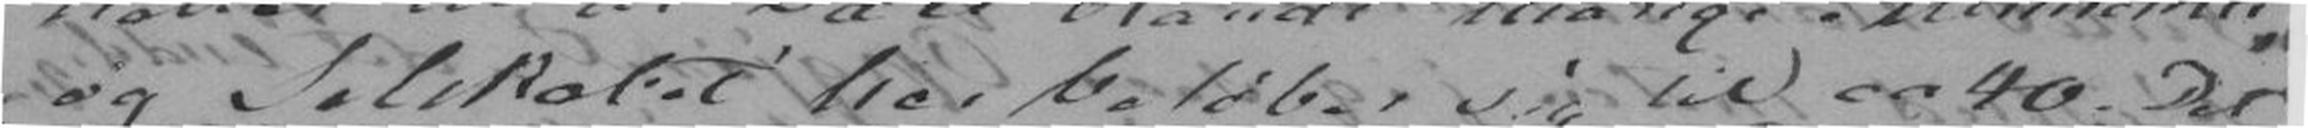og Selskabet her beløber sig til ca 40. Det
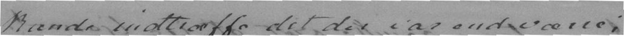kunde indtræffe det der var end værre
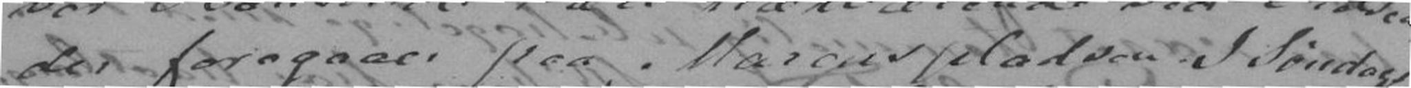der foregaar paa Marenspladsen. I Søndags
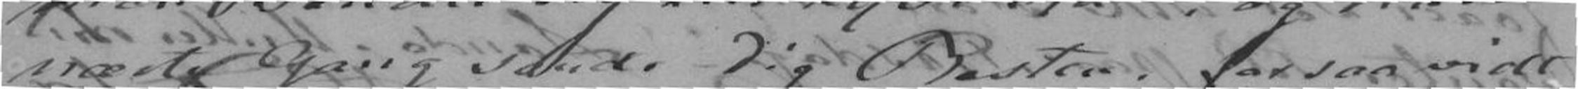næste Gang sende Dig Ræsten, forsaavidt
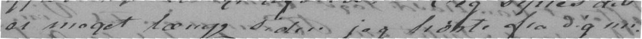er meget længe siden jeg hørte fra Dig nu,
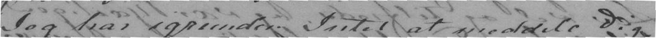Jeg har igrunden Intet at meddele Dig
